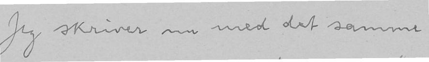Jeg skriver nu med det samme
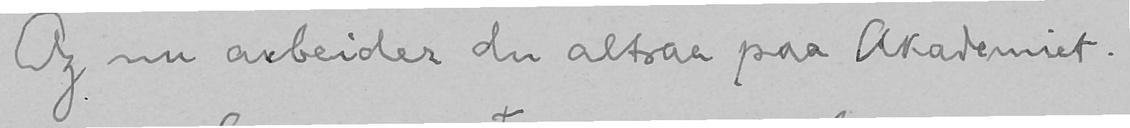Og nu arbeider du altsaa paa Akademiet.
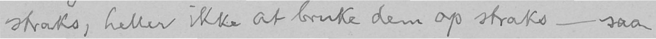straks, heller ikke at bruke dem op straks - saa
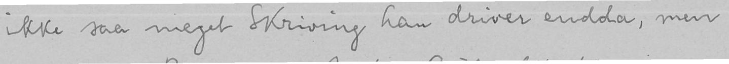ikke saa meget skriving han driver endda, men
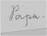Papa.
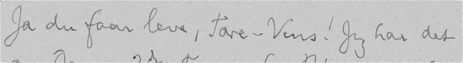Ja du faar leve, Tore-Vens! Jeg har det
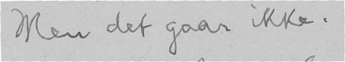Men det gaar ikke.
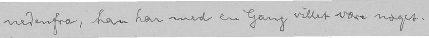nedenfra, han har med en Gang villet være noget.
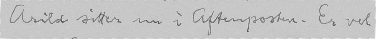Arild sitter nu i Aftenposten. Er vel
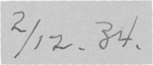2/12. 34.
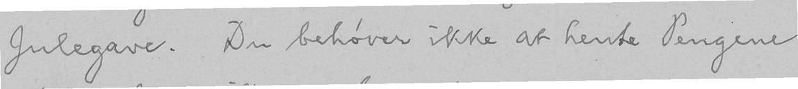Julegave. Du behøver ikke at hente Pengene
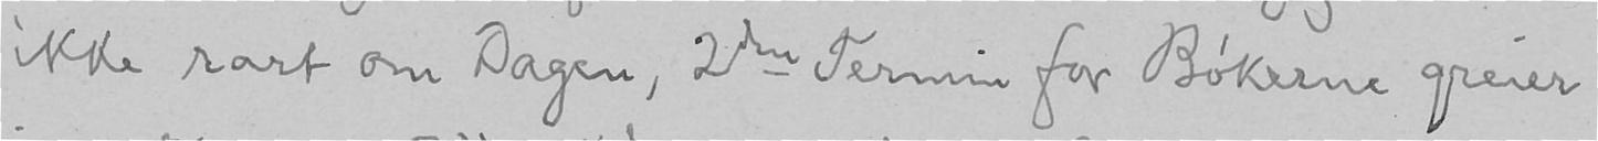ikke rart om Dagen, 2den Termin for Bøkerne greier
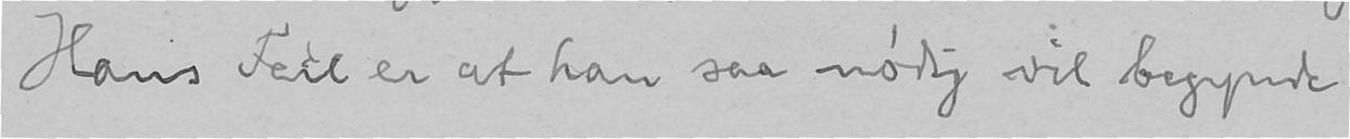Hans Feil er at han saa nødig vil begynde
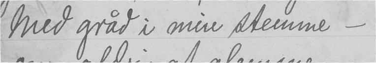Med gråd i min stemme -
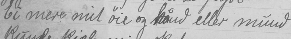Ei mere mit øie og hånd eller mund
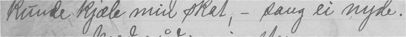kunde kjæle min skat, - sang ei nyde.
